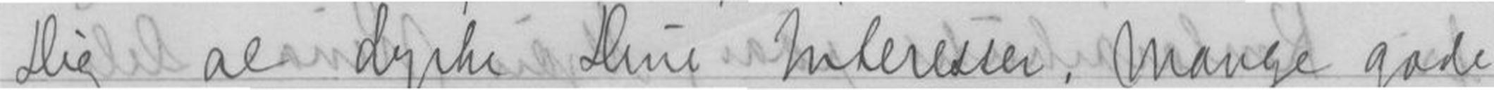Dig at dyrke Dine Interesser. Mange gode
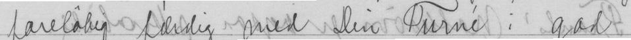foreløbig færdig med Din Turnè i god
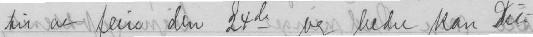til at feire den 24de og bedre kan Du
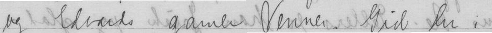og Edvards gamle Venner. Gid du i
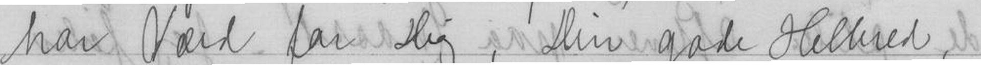har Værd for Dig, Din gode Helbred,
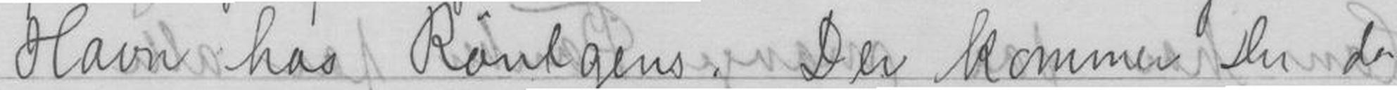Havn hos Röntgens. Der kommer Du da
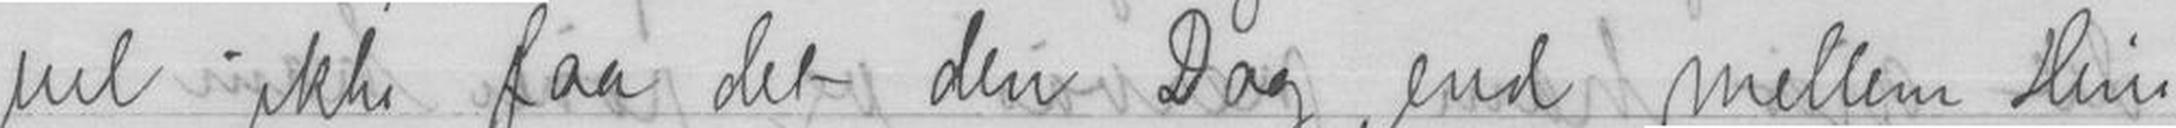vel ikke faa det den Dag, end mellem Dine
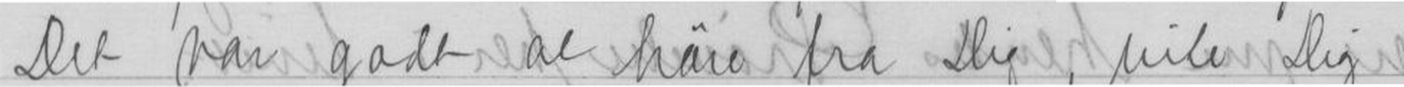Det var godt at høre fra Dig, vide Dig
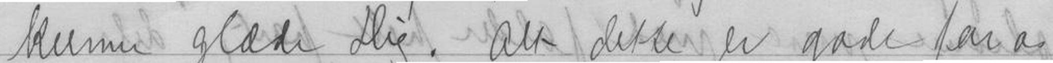kunne Glæde Dig. Alt Dette er godt for os
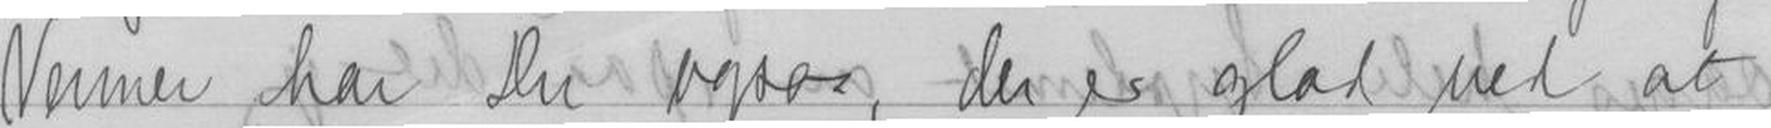Venner har Du ogsaa, der er glad ved at
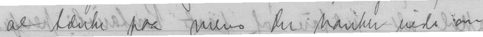at tænke paa, mens Du vandrer vide om
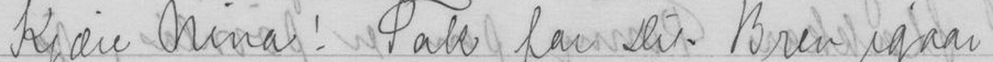Kjære Nina! Tak for Dit Brev igaar
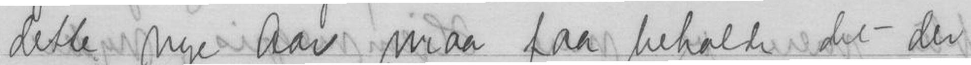dette nye Aar maa faa beholde det der
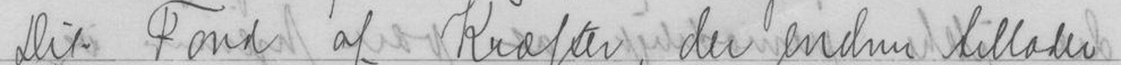Dit Fond af Kræfter, der endnu tillader
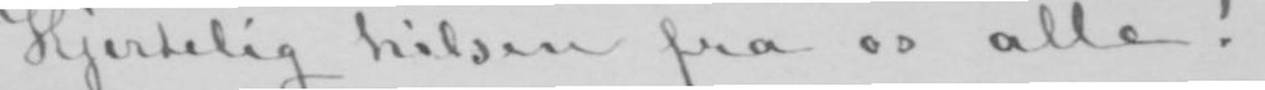Hjertelig hilsen fra os alle!
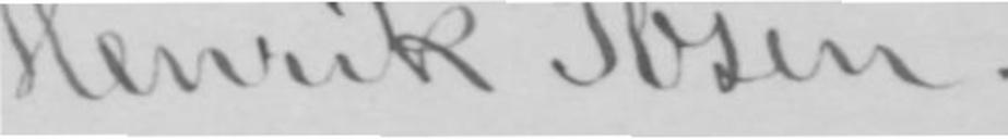Henrik Ibsen.
